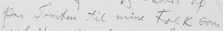paa Tomten til mine Folk som
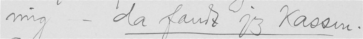mig - da fandt jeg Kassen.
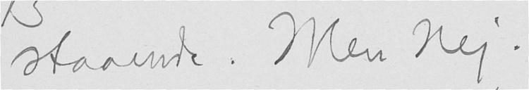staaende. Men Nej.
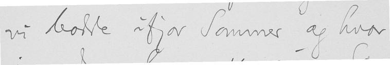vi bodde ifjor Sommer og hvor
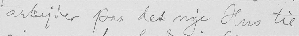arbejder paa det nye Hus til
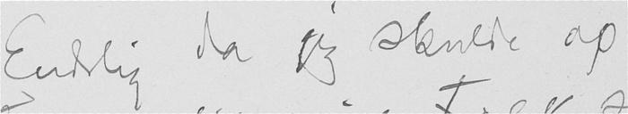Endelig da jeg skulde op
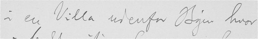i en Villa udenfor Byen hvor
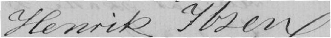Henrik Ibsen
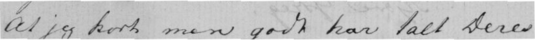At jeg kort men godt har talt Deres
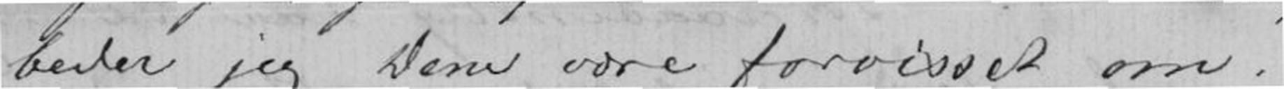beder jeg Dem være forvisset om.
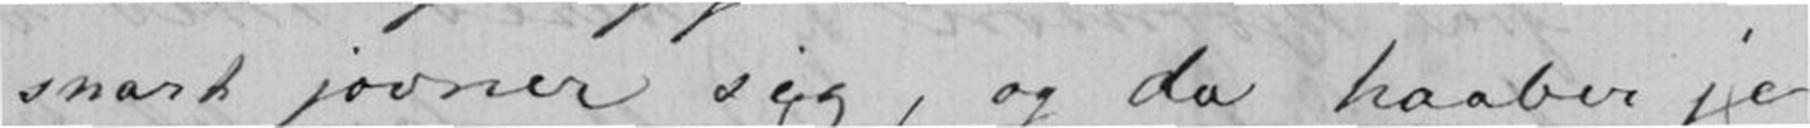snart jevner sig, og da haaber jeg
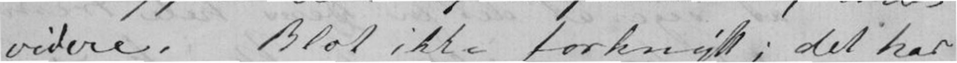videre. Blot ikke forknytt; det har
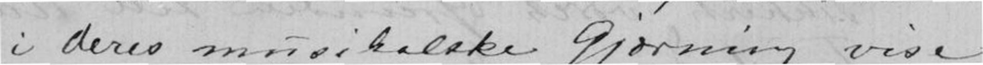i Deres musikalske Gjørning vise
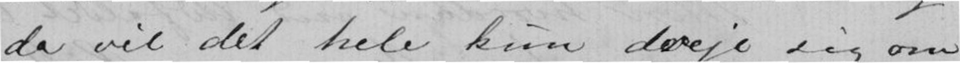da vil det hele kun dreje sig om
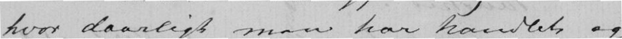hvor daarligt man har handlet og
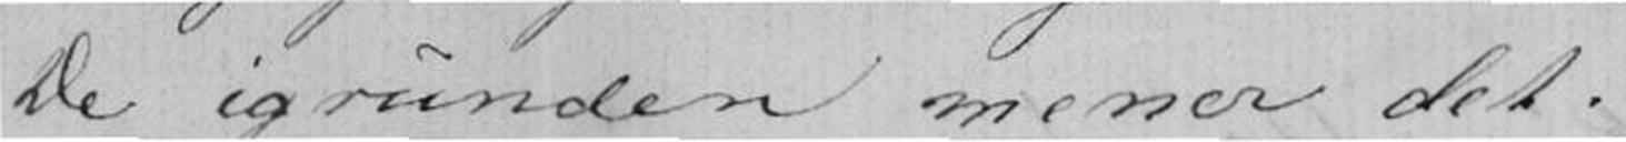De igrunden mener det.
